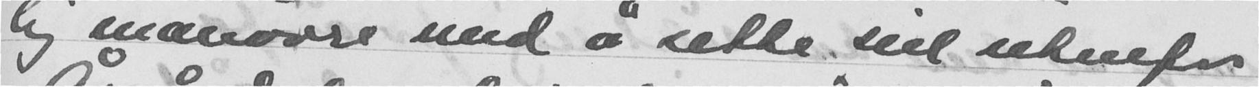lige manøvre med å sette seil utenfor
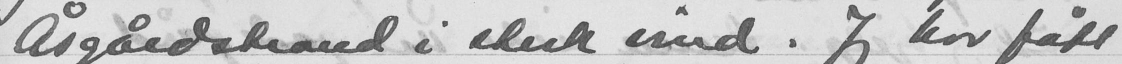Åsgårdstrand i slik vind. Jeg har fått
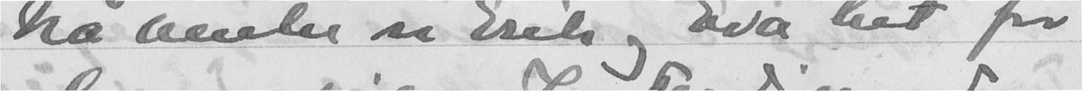Nå venter vi Erik og Eva hit for
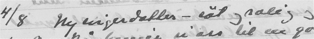4/8 Ny svigerdatter - søt og rolig og
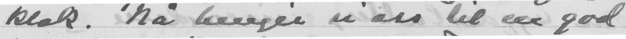klok. Nå henger vi oss til en god
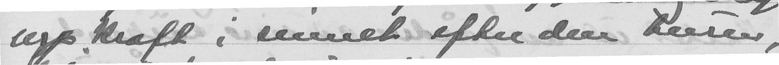ny kraft i sinnet efter den turen,
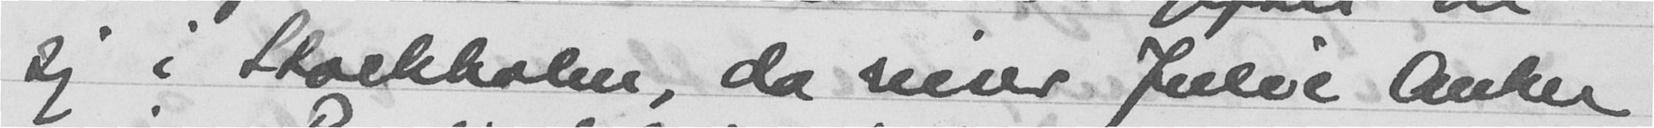sig i Skochholm, da reiser Julie Anker
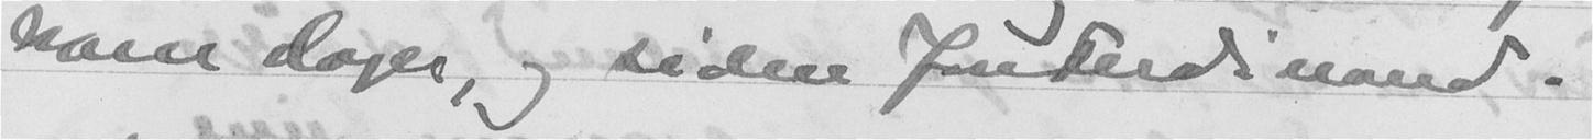noen dager, og siden Far Ferdinand.
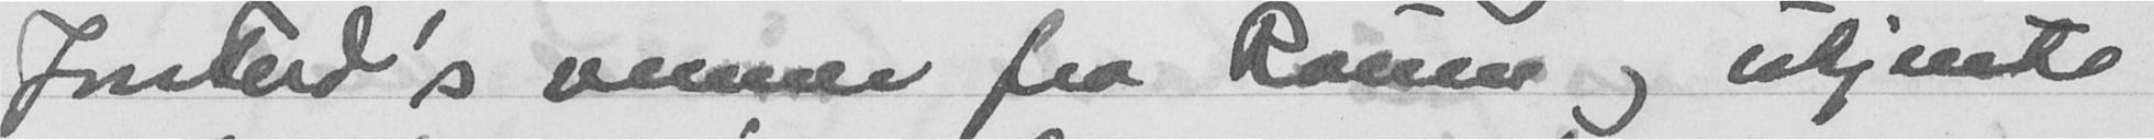FruFerd's venner fra Rauen og ukjente
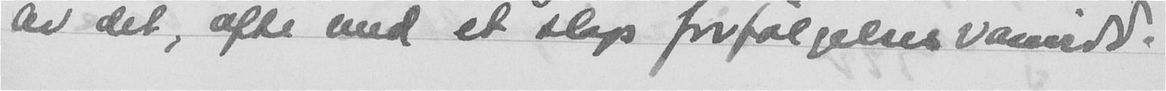av det, ofte med et slags forfølgelsesvanvidd.
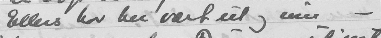Ellers har her vært ut og inn -
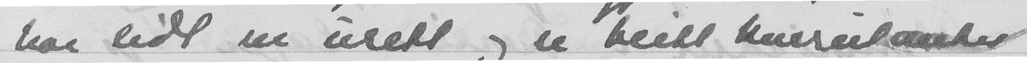har lidt en usett og se blitt kursutanter
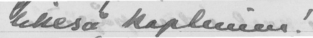likeså kapteinen.
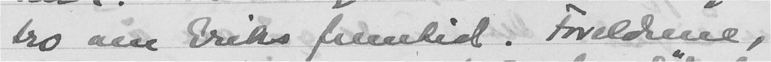tro om Eriks fremtid. Foreldrene,
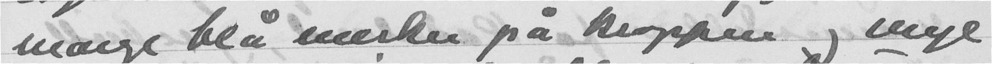mange blå merker på kroppen og mye
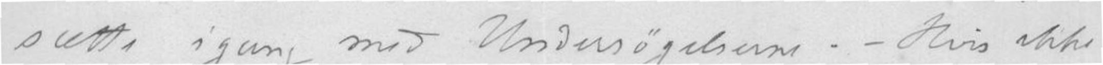sætte igang med Undersøgelserne. - Hvis ikke
